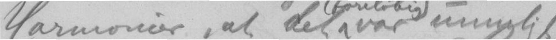Harmonier, at det var umuligt
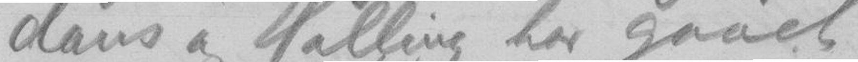dans og Halling har gaaet
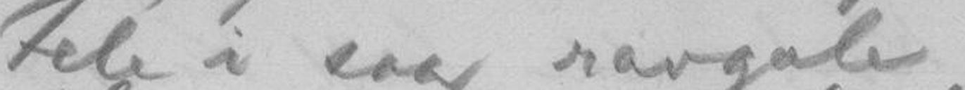Fele i saa ravgale
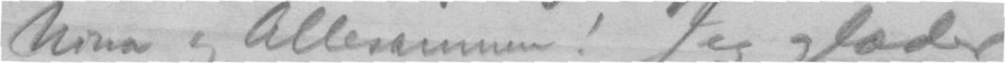Nina og Allesammen! Jeg glæder
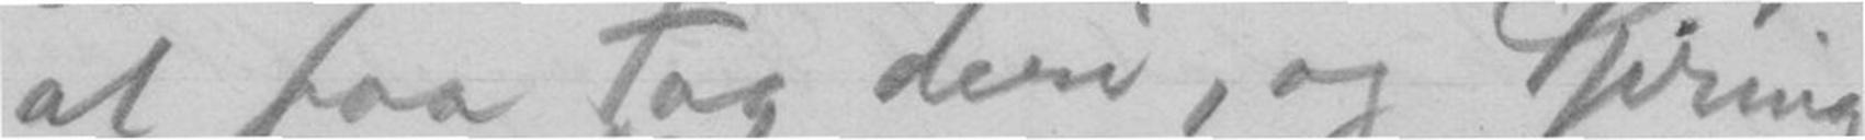at faa Tag deri, og Spring-
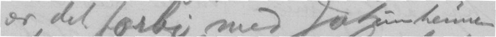er det forbi med Jotunheimen
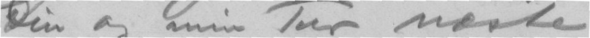Din og min Tur næste
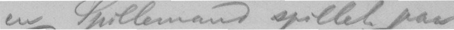en Spillemand spillet paa
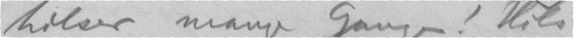hilser mange Gange! Hils
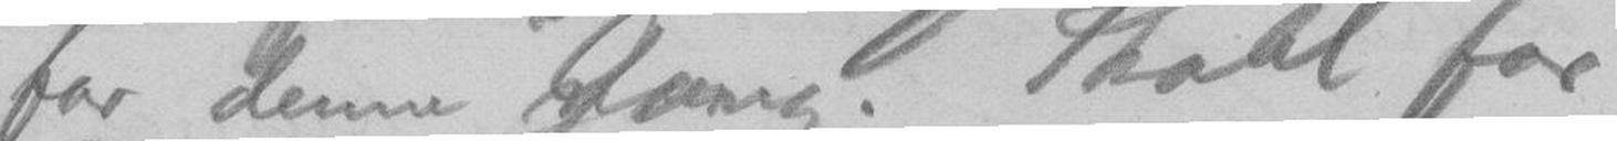for denne Gang. Skaal for
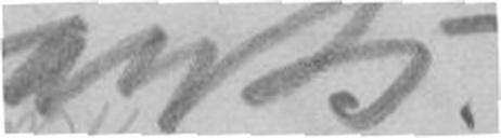Frants
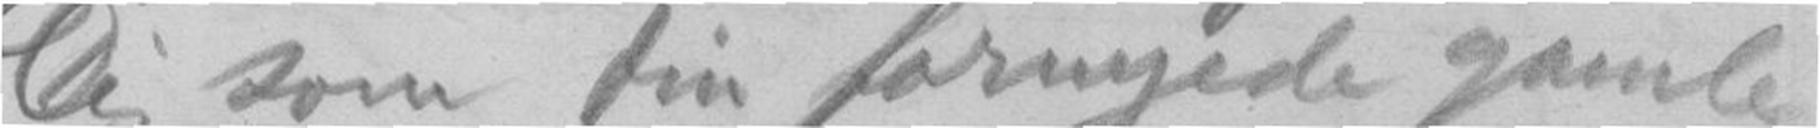Dig som Din fornyede gamle
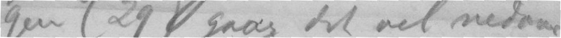gen (29) gaar det vel nedover
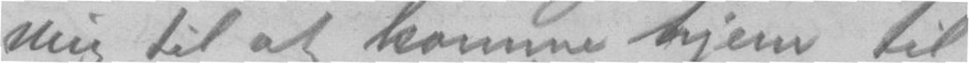mig til at komme hjem til
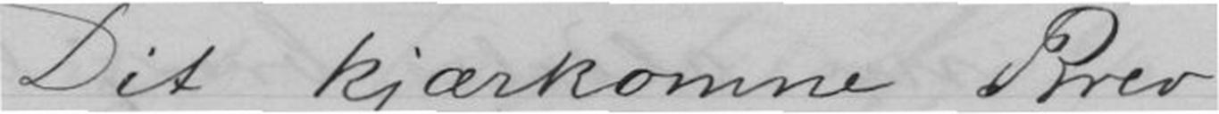Dit kjærkomne Brev
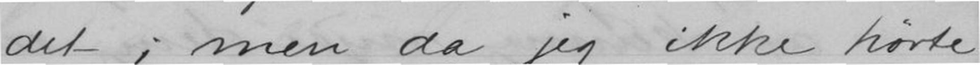det; men da jeg ikke hørte
